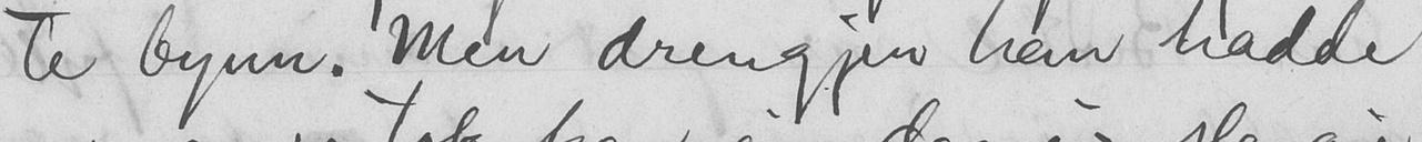te bynn. Men drengjen han hadde
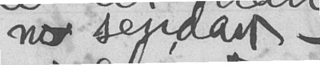no sendast
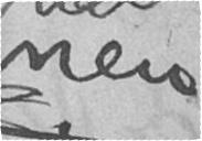men
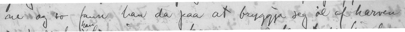ne og so fann han da paa at bryggja seg øl af harven
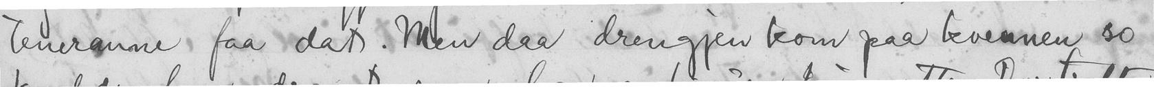teneranne faa dat. Men daa drengjen kom paa kvennen so
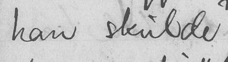han skulde
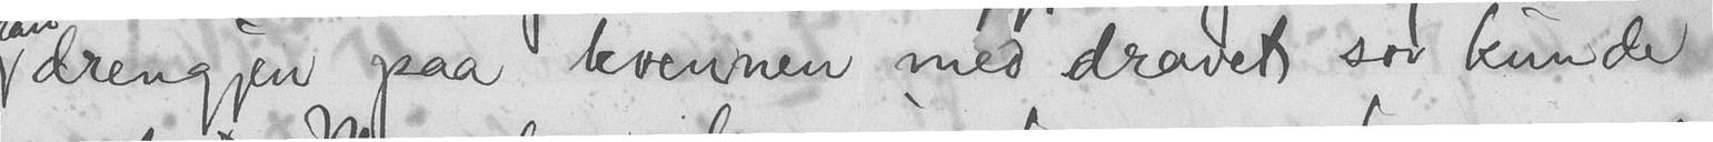drengjen paa kvernen med dravet so kunde
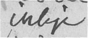inkje
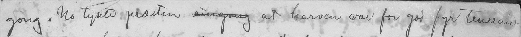gong. No tykte præsten  at harven var for god fyr teneran-
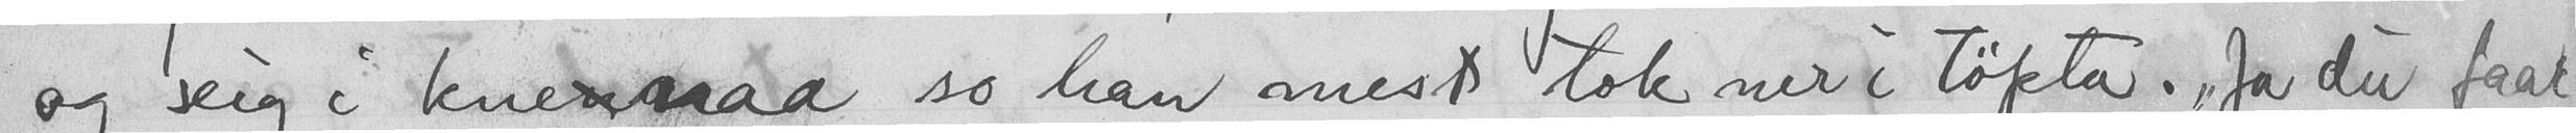og seig i knenaa so han mest tok ner i tøpta. "Ja du faar
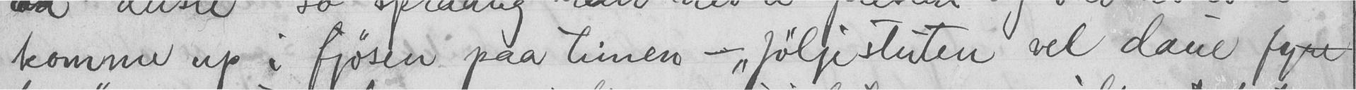komme up i fjøsen paa timen - "jøljestuten vel daue fyre
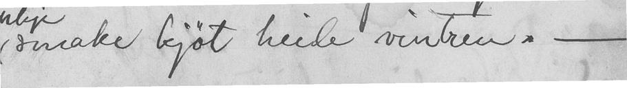smake kjøt heile vintren. -
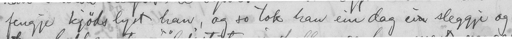fengje kjødslyst han, og so tok han ein dag ei sleggje og
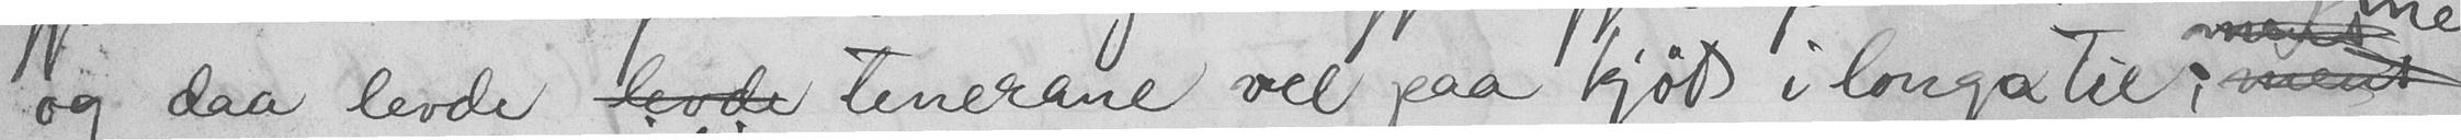og daa levde  tenerane vel paa kjøt i longa tie;
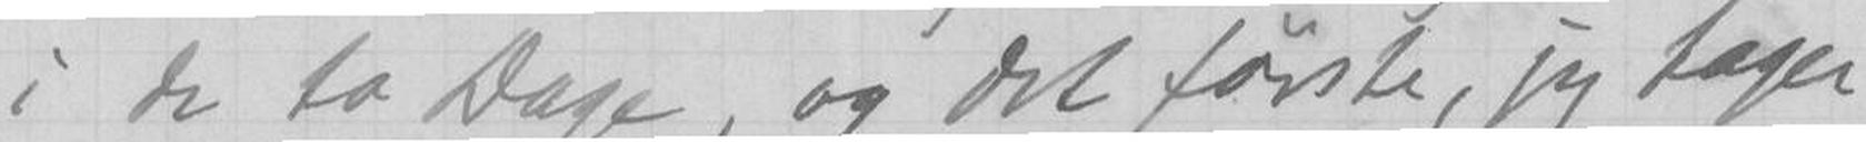i de to Dage, og det første, jeg tager
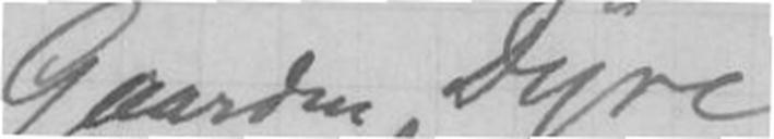Gaarden Dyre
